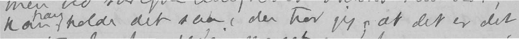kan han holde det saa, der tror jeg, at det er det
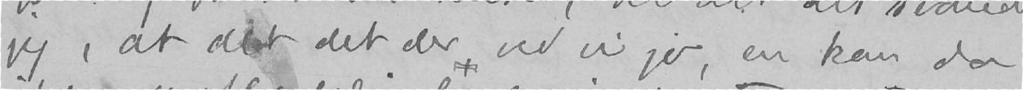jeg, at alt det det , ved vi jo, en kan da
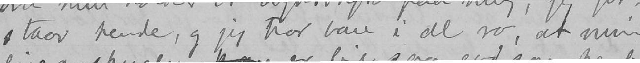staar hende, og jeg tror bare i all ro, at min
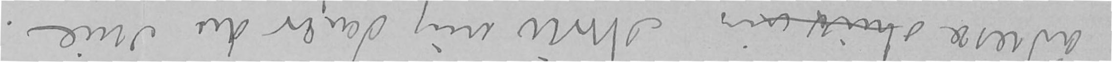adresse skriv vir skriv mig den, er du snil.
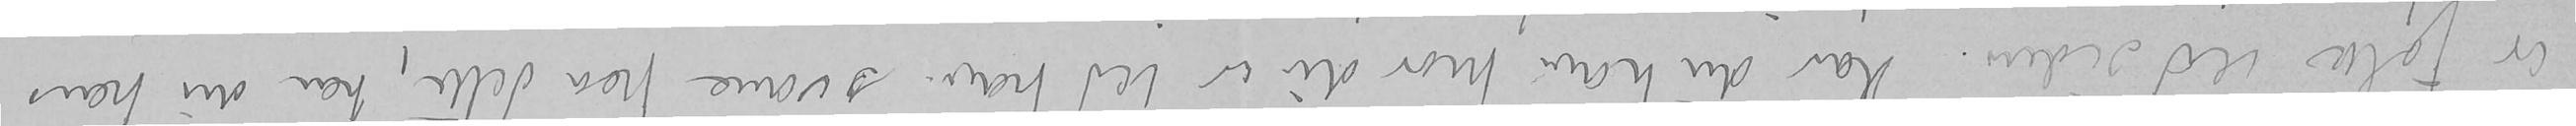er folk ved siden. Har du ham hvor du er bed ham svare paa dette, har du hans
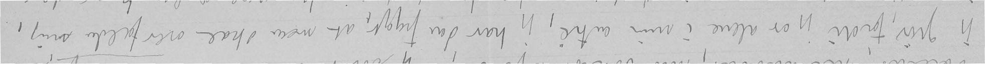jeg giør, fordi jeg er alene i min entre, jeg har den frygt, at noen skal overfalde mig,
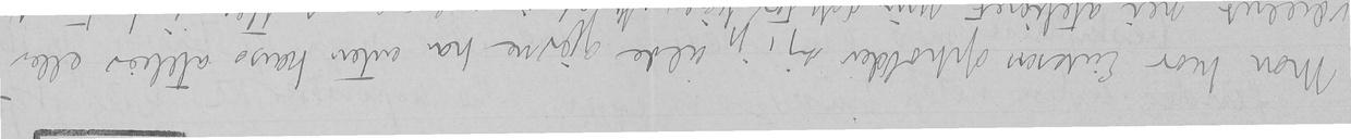Mon hvor Eriksen opholder sig, jeg vilde gjerne ha enten hans atelier eller
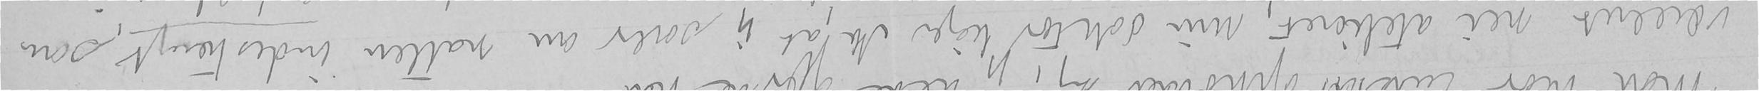værelset, nei atelieret, min doktor liger ikke, at jeg sover om natten indestængt, som
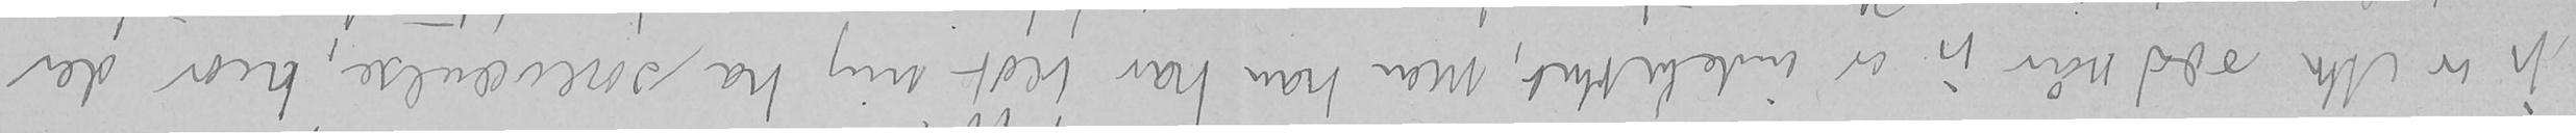jeg er ikke ræd når jeg er indelukket, men han har bedt mig ha soveværelse, hvor der
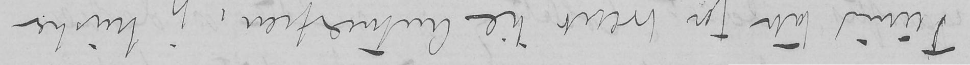Tusind tak for brevet til Antwærpen, jeg husker
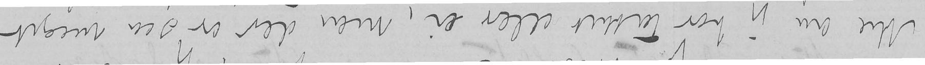ikke om jeg har takket eller ei, men der er saa meget
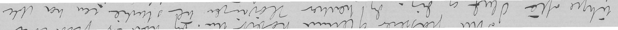uhyre ofte Oluf og dig. Jeg tænker Høvringen til studie men har ikke
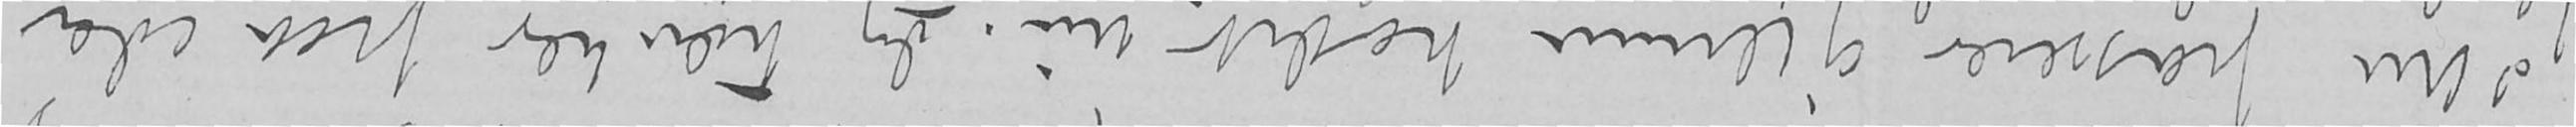som passerer gjennem hodet nu. Jeg tænker paa eder
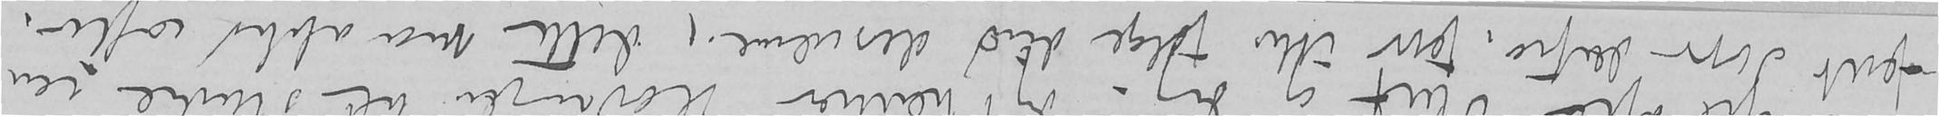faat svar derfra, faar ikke følge did desværre, dette maa aftid ioften.
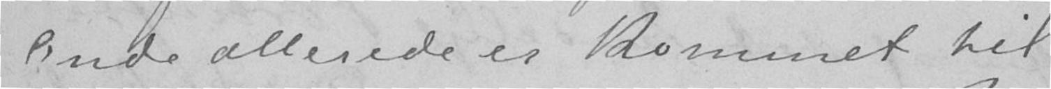Onde allerede er kommet til
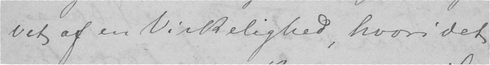vet af en Virkelighed, hvori det
